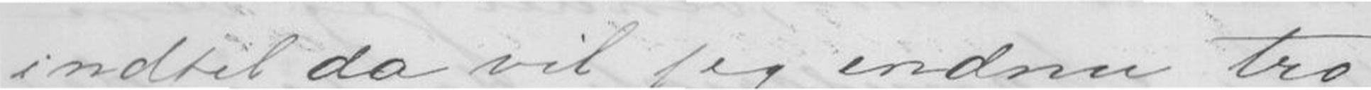indtil da vil jeg endnu tro
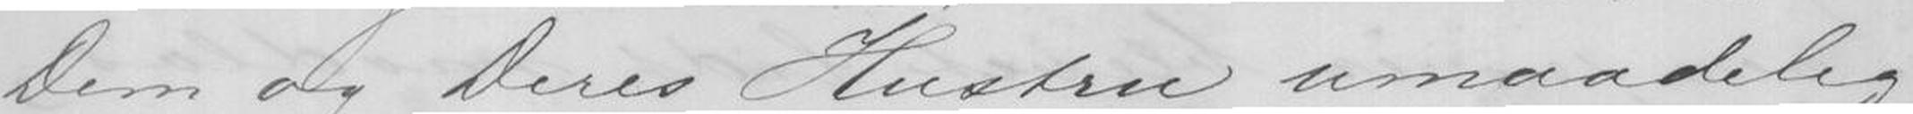Dem og Deres Hustru umaadelig
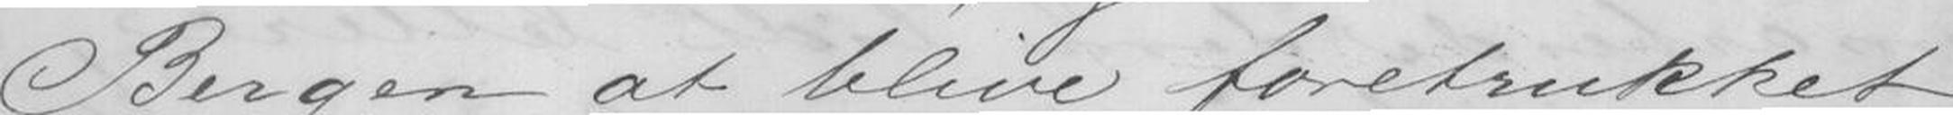Bergen at blive foretrukket
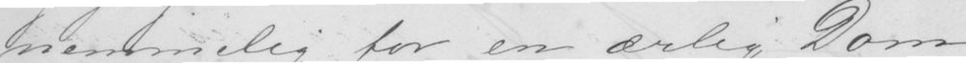nemmelig for en ærlig Dom,
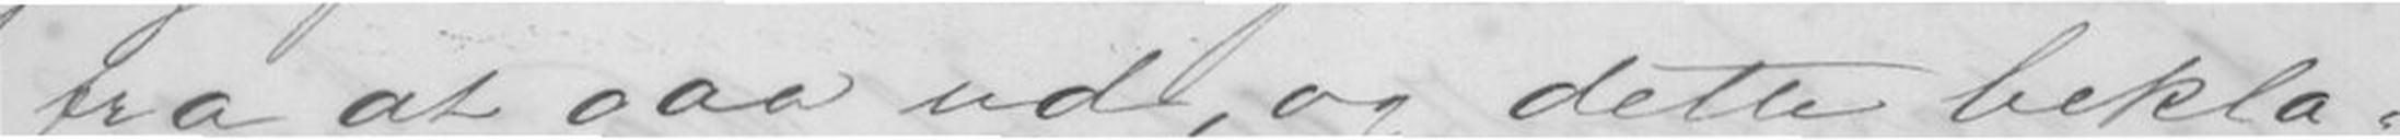fra at gaa ud, og dette bekla-
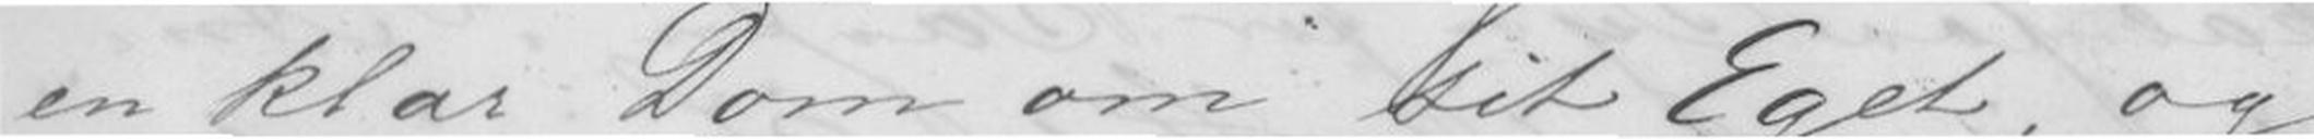en klar Dom om sit Eget, og
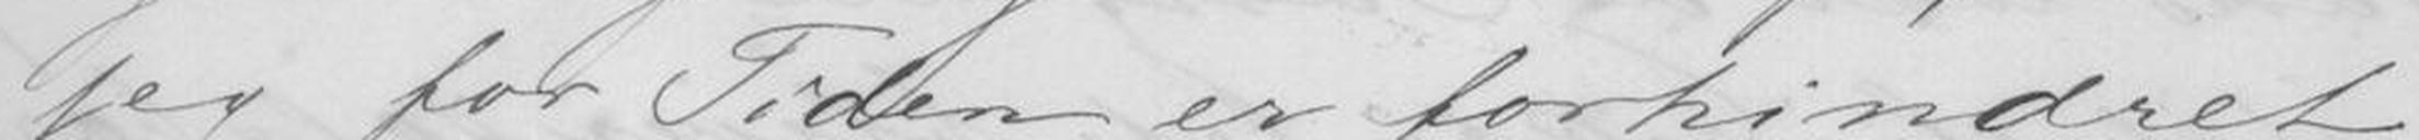jeg for Tiden er forhindret
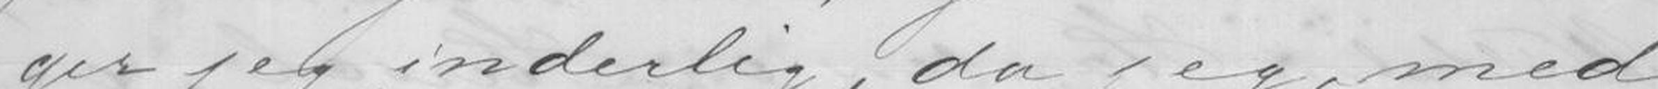ger jeg inderlig, da jeg, med
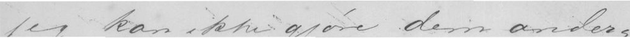jeg kan ikke gjøre dem ander-
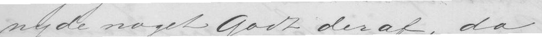nyde noget Godt derat, da
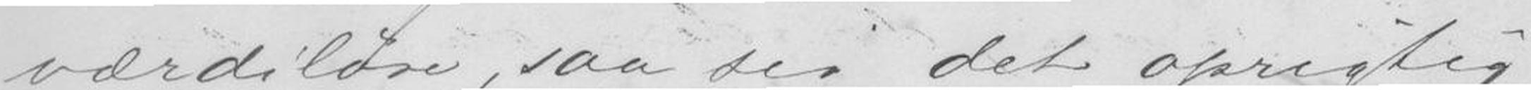værdiløse, saa sig det oprigtig,
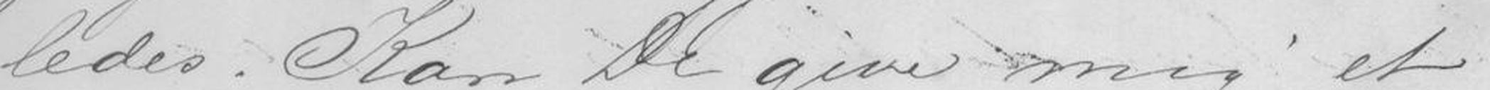ledes. Kan De give mig et
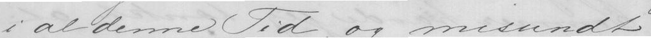i al denne Tid, og misundt
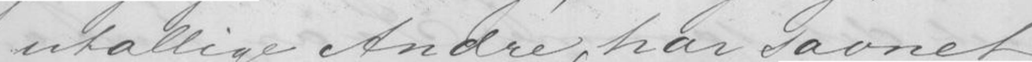utallige Andre, har savnet
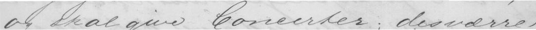og skal give Concerter; desværre,
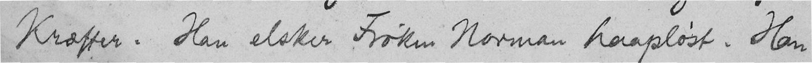Kræfter. Han elsker Frøken Norman haapløst. Han
